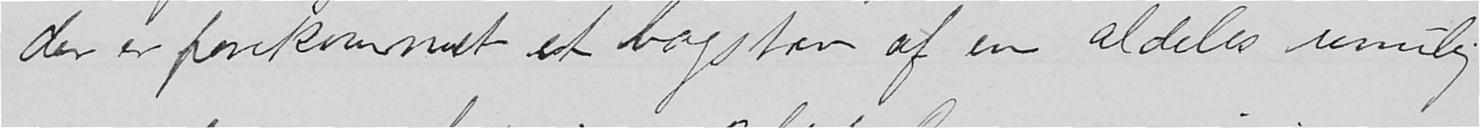der er forekommet et bogstav af en aldeles umulig
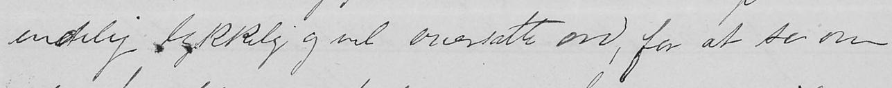endelig lykkelig og vel oversatte ord, for at se om
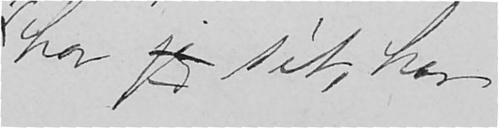har jeg sét, har
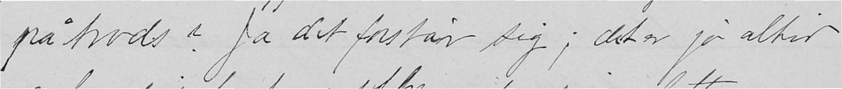på trods? Ja det forstår sig; det er jo altid
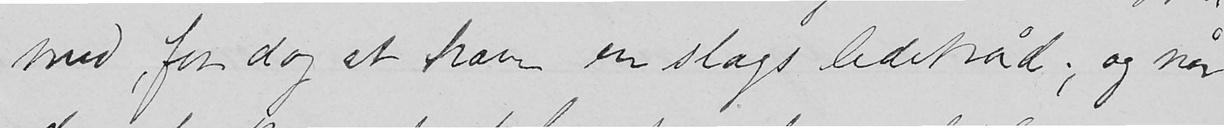med, for dog at have en slags ledetråd; og når
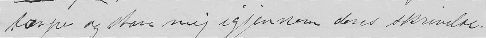tærpe og stave mig igjennem deres skrivelse.
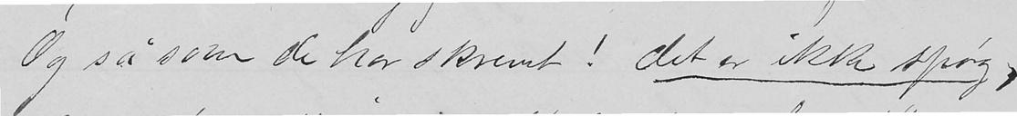Og så som de har skrevet! Det er ikke spøg,
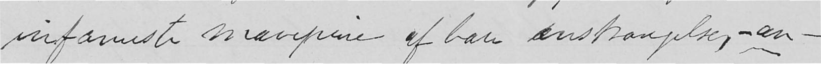infameste mavepine af bare anstrængelse - an-
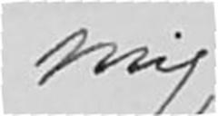mig
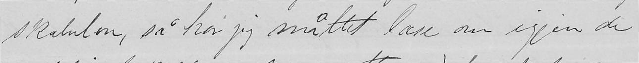skabelon, så har jeg måttet læse om igjen de
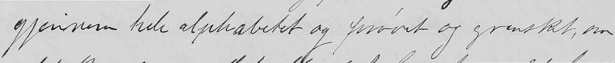gjennem hele alphabetet og prøvet og gransket, om
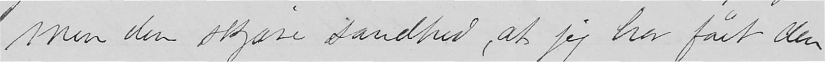men den skjære sandhed, at jeg har fået den
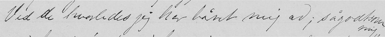Ved de hvorledes jeg har båret mig ad; sågodtsom
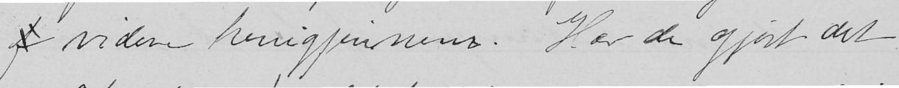f videre henigjennem. Har de gjort det
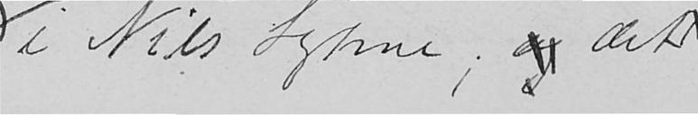i Nils Lyhne; og det
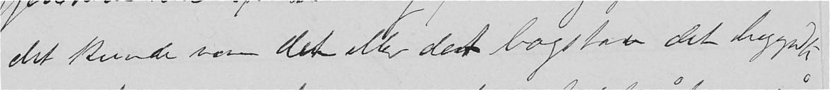det kunde være det eller det bogstav det begyndte
